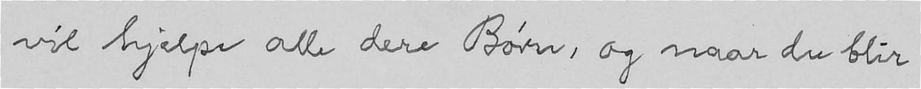vil hjelpe alle dere Børn, og naar du blir
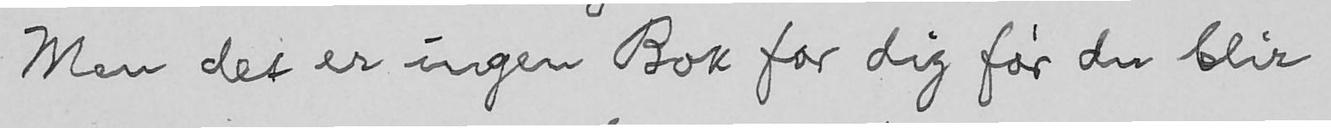Men det er ingen Bok for dig før du blir
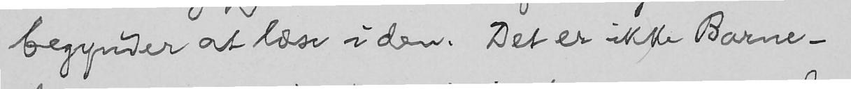begynder at læse i den. Det er ikke Barne-
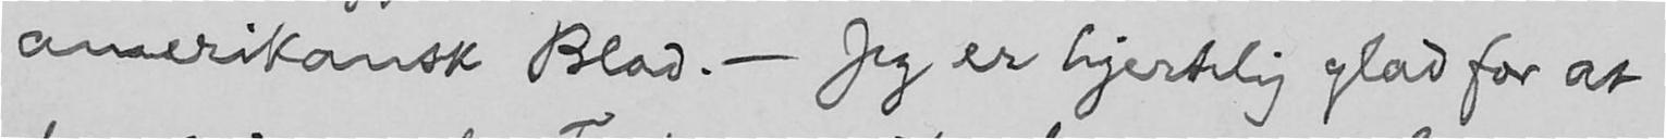amerikansk Blad. - Jeg er hjertelig glad for at
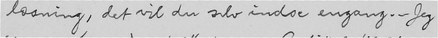læsning, det vil du selv indse engang. - Jeg
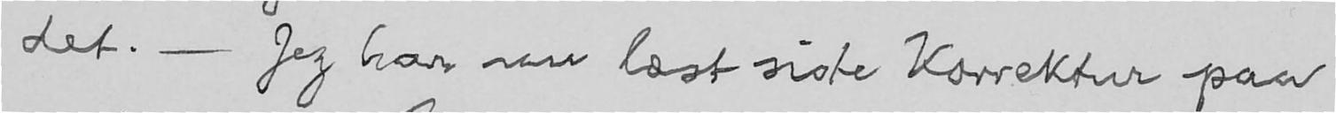det. - Jeg har nu læst siste Korrektur paa
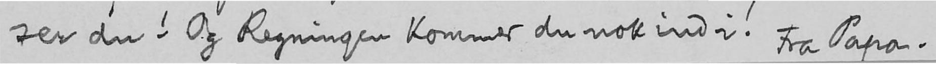ser du! Og Regningen kommer du nok ind i! Fra Papa.
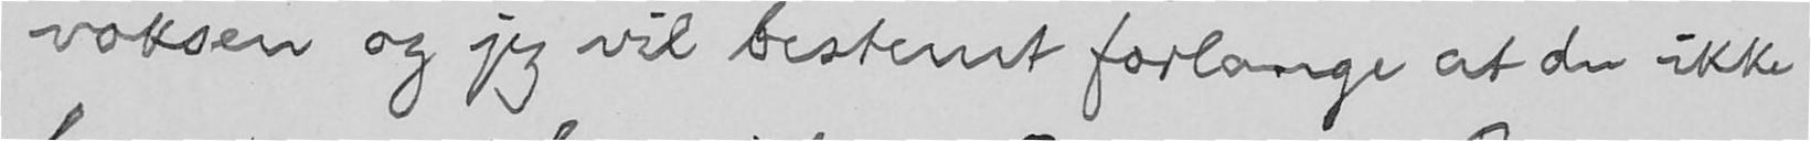voksen og jeg vil bestemt forlange at du ikke
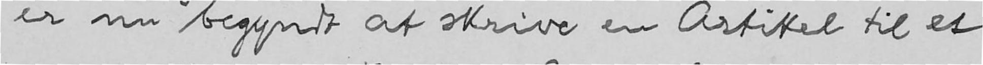er nu begyndt at skrive en Artikel til et
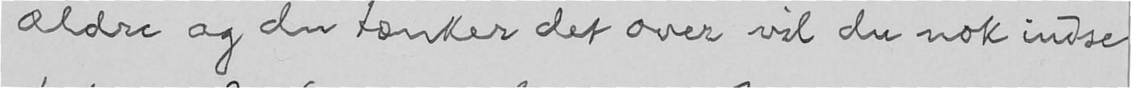ældre og du tænker det over vil du nok indse
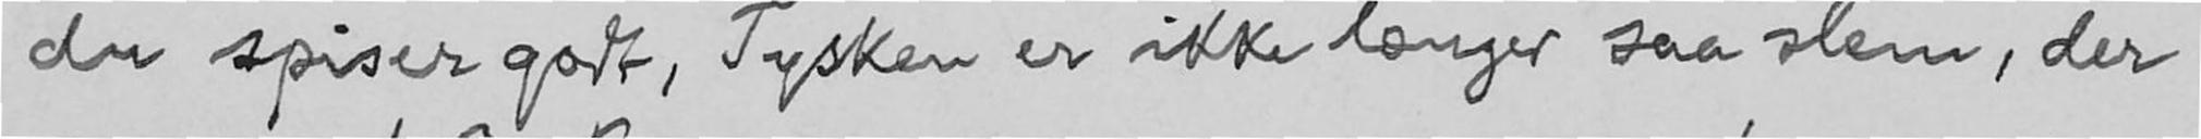du spiser godt, Tysken er ikke længer saa slem, der
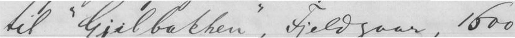til "Gjilbakken", Fjeldgaar, 1600
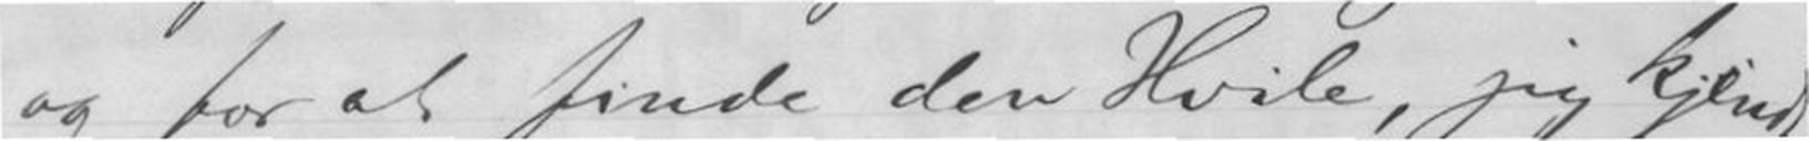og for at finde den Hvile, jeg kjendte
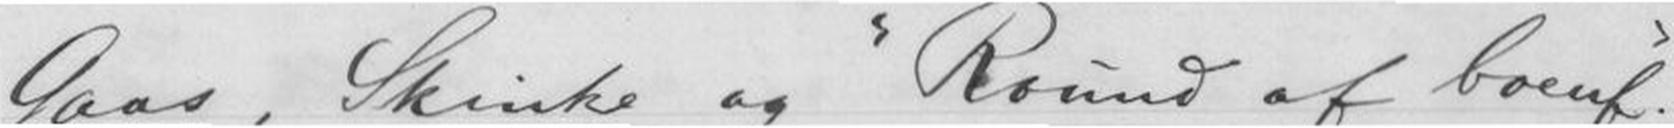Gaas, Skinke og "Round of boeuf".
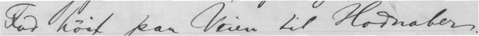Fod høit paa Veien til Hodnaber.
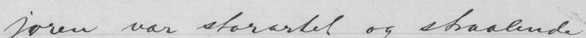joren var storartet og straalende
